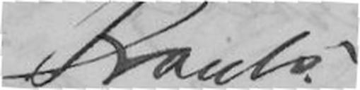Frants.
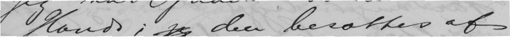Glands; je den besattes af
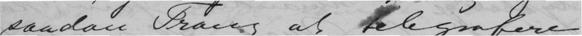saadan Trang at telegrafere
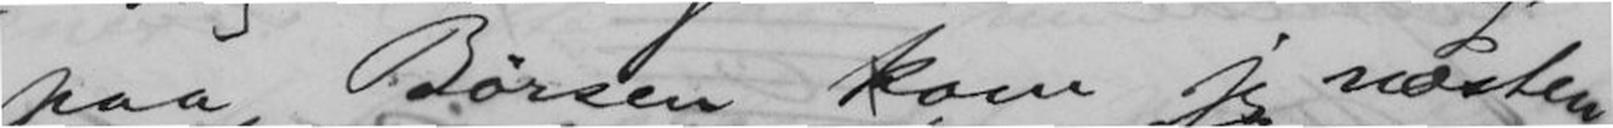og paa Børsen kom jeg næsten
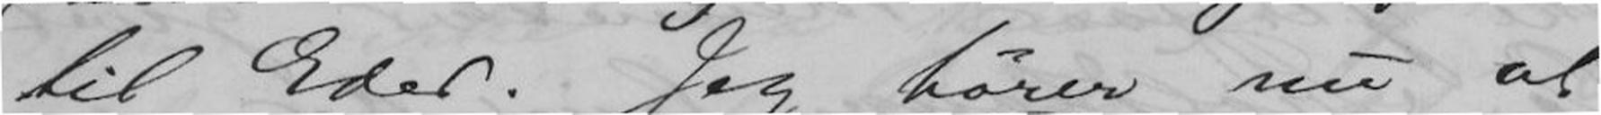til Eder. Jeg hører nu at
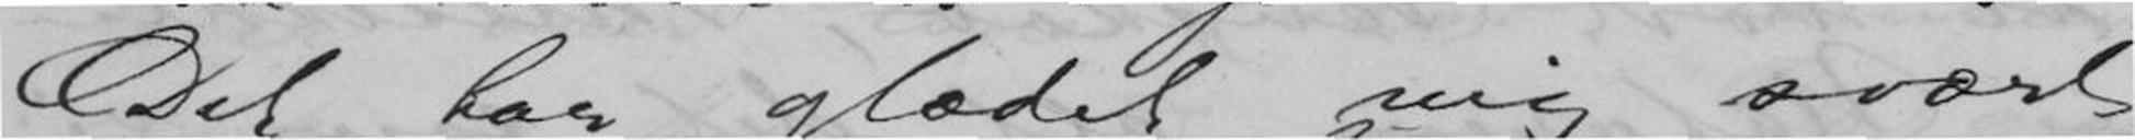Det har glædet mig svært
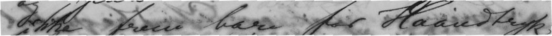ikke frem bare for Haandtryk
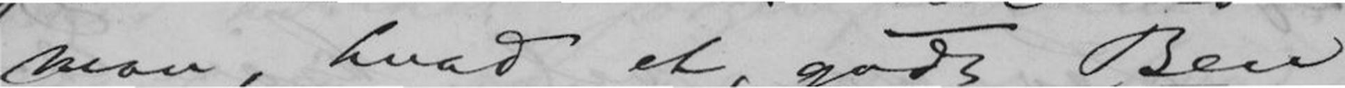man, hvad et godt Ben
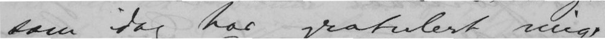som idag har gratuleret mig,
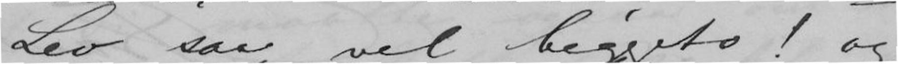Lev saa vel beggeto! og
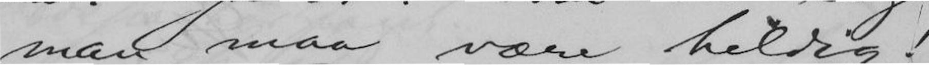man maa være heldig!
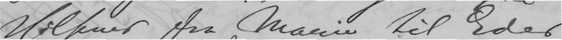Hilsener fra Marie til Eder
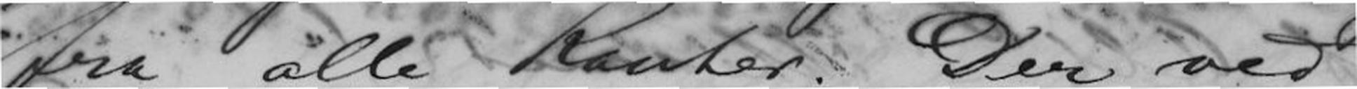fra alle Kanter. Der ved
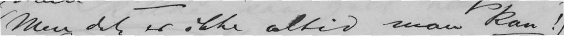(Men det er ikke altid man kan!)
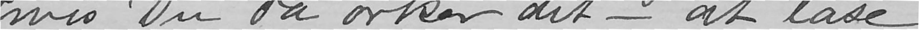hvis Du da orker det- at læse
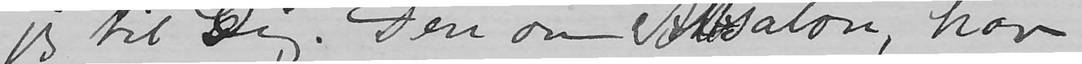jeg til Dig. Den om Absalom, har
